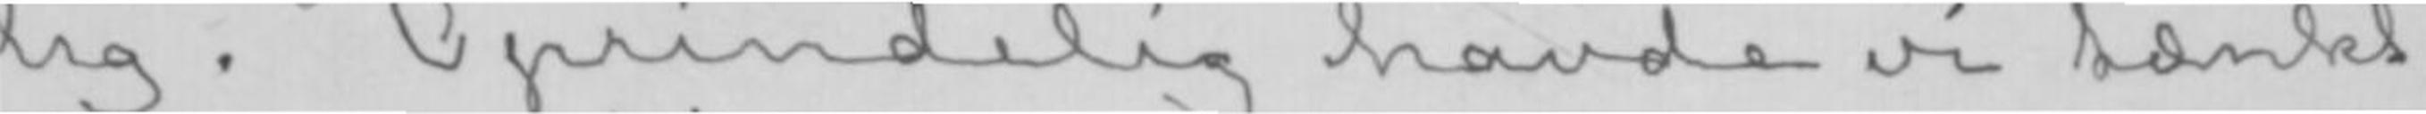lig. Oprindelig havde vi tænkt
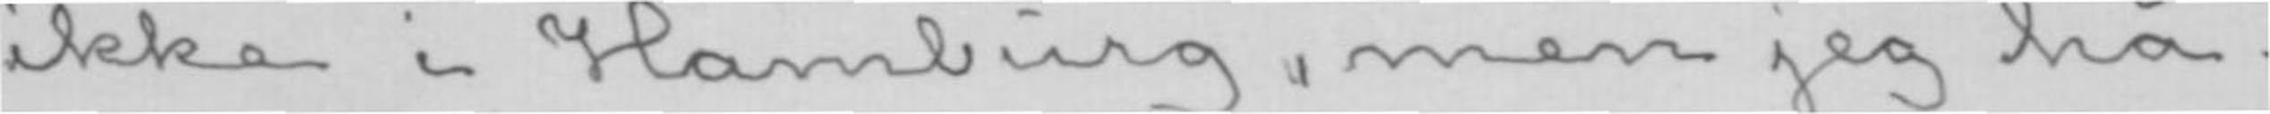ikke i Hamburg, men jeg hå-
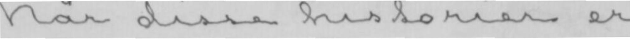Når disse historier er
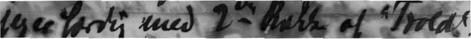jeg er færdig med 2den Række af Trold!
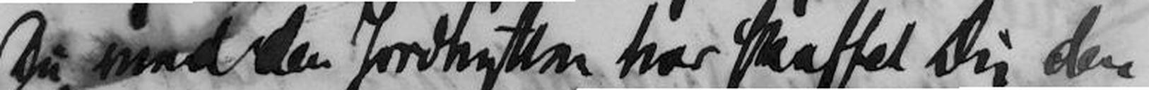Du med at den Jordhytten har skaffet Dig den
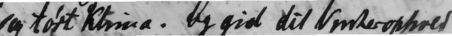og tørt Klima. Og gid dit Vinterophold
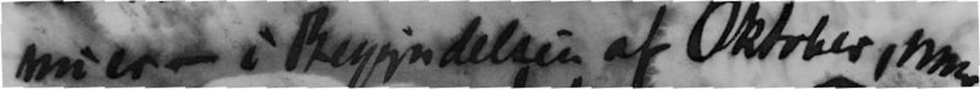nu var i Begyndelsen af Oktober, men
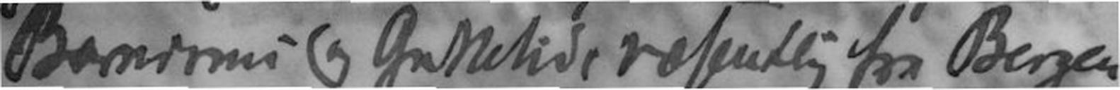Barndoms og guttetid, væsentlig fra Bergen.
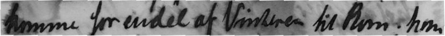komme for endel af Vinteren til Rom; han
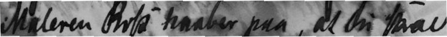Maleren Ross haaber paa at Du skal
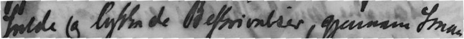fulde og lytter de Beskrivelser, gjennem Smaa
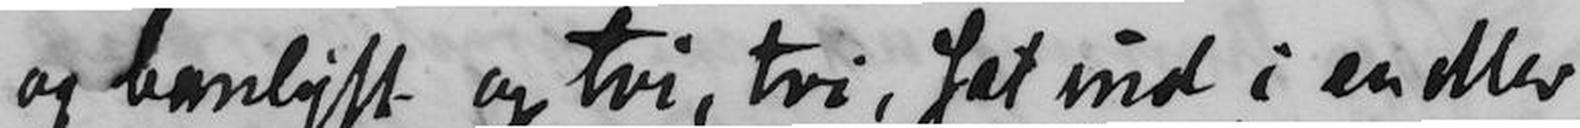og banlyst og tvi, tvi, sat ind i en eller
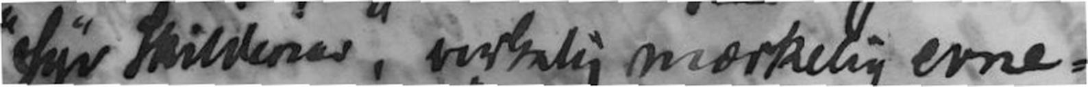"syv Skildener" virkelig mærkelig evne-
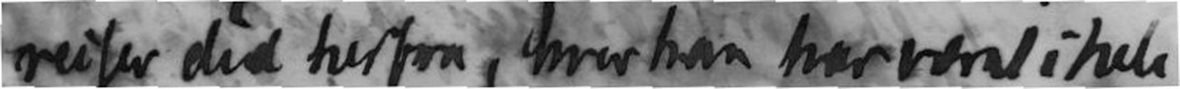reiser did herfra, hvor han har vært i hele
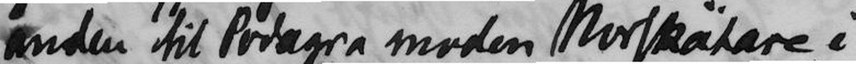anden til Podagra moden Norskätare i
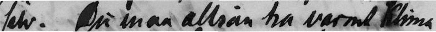selv. Du maa altsaa ha varmt Klima
